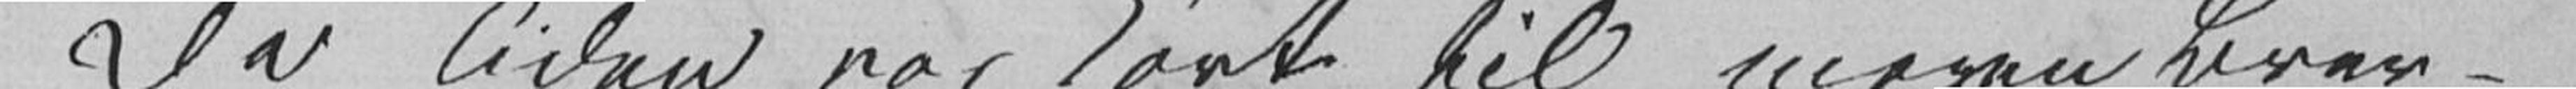Da Tiden var kort til megen Brev¬
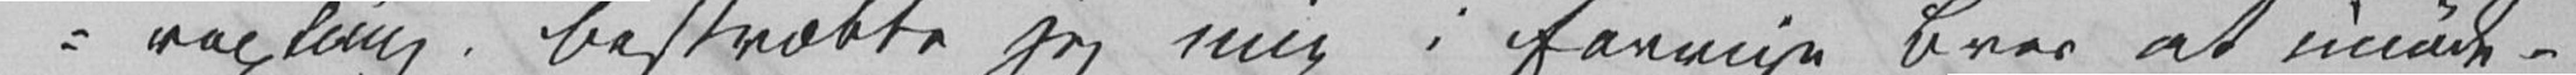vexling, bestræbte jeg mig i forrige Brev at imøde¬
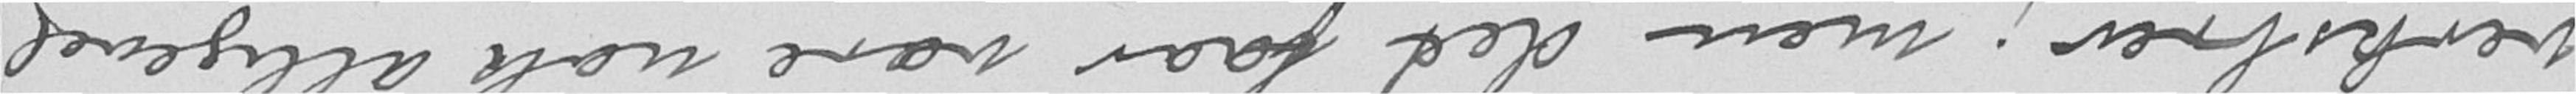verksbrev; men det faar være nok alligevel,
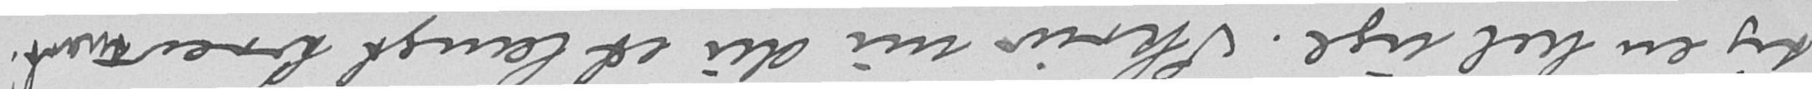sig en hel uge. Skriv nu du et langt brev snart!
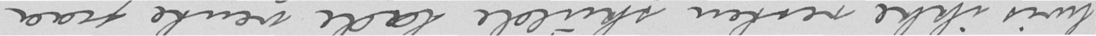hvis ikke resten skulde lade vente paa
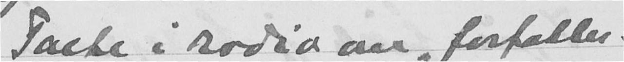Talte i radio om "fortatter-
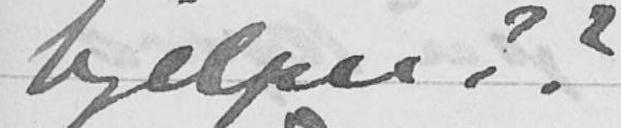hjelpes??
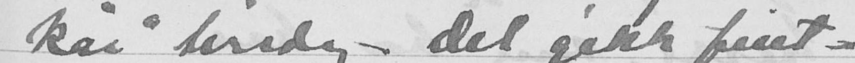kår" tirsdag - Det gikk fint.
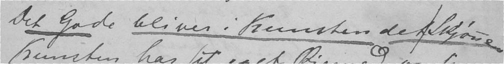Det Gode bliver i Kunsten det skjønne.
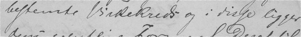bestemte Virkekreds og i disse ligger
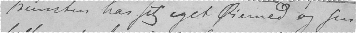Kunsten har sit eget Øiemed og sin
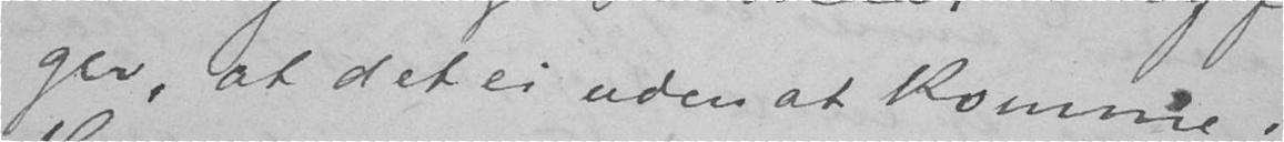ger, at det ei uden at komme i
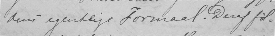dens egentlige Formaal. Deraf føl-
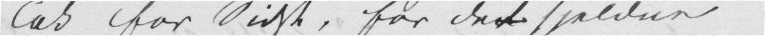Tak for Sidst, for det sjeldne
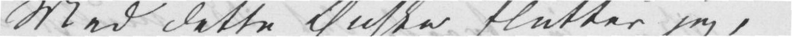Med dette Ønske slutter jeg,
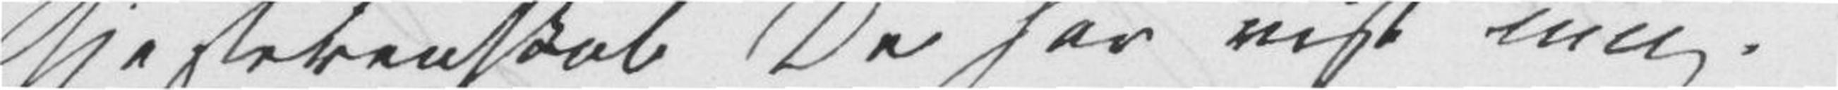Gjestevenskab De har vist mig.
